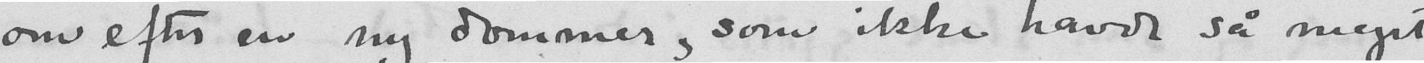om eften en ny dommer, som ikke havde så meget
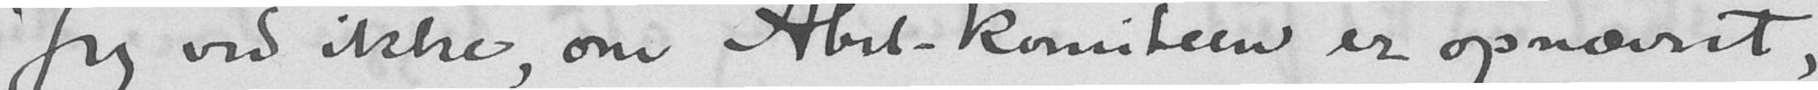Jeg ved ikke, om Abel-komiteen er opnævnt,
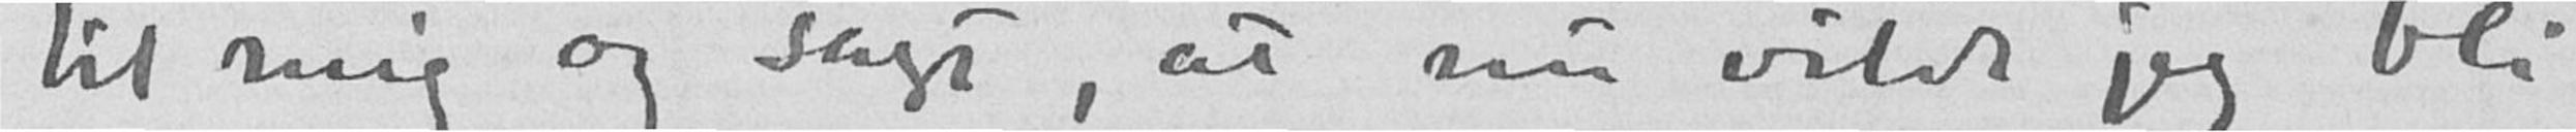til mig og sagt, at nu vilde jeg bli
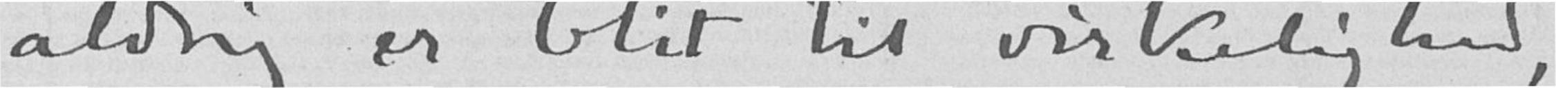aldrig er blit til virkelighed,
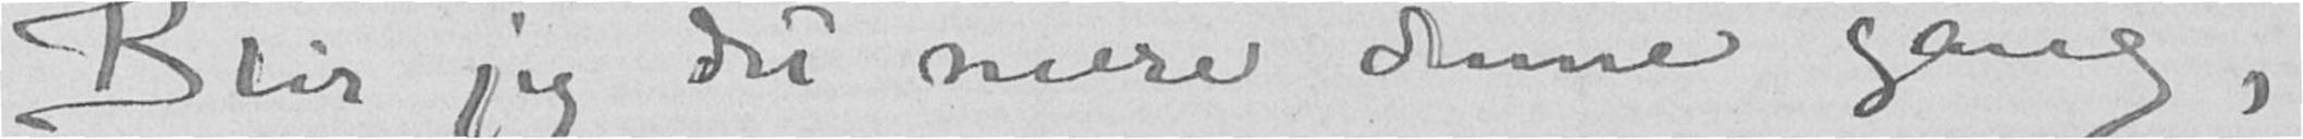Blir jeg det mere denne gang,
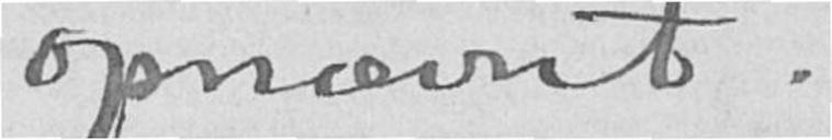opnævnt.
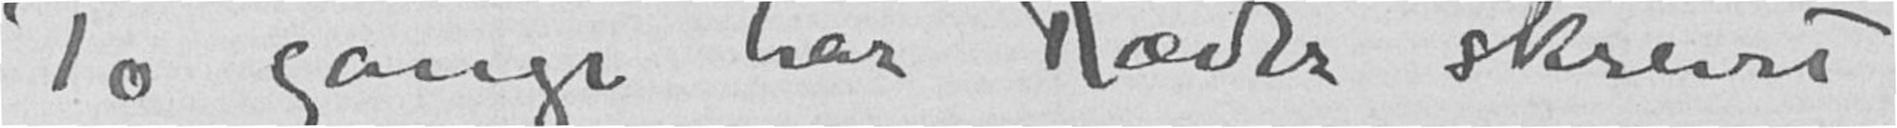To ganger har Ræder skrevet
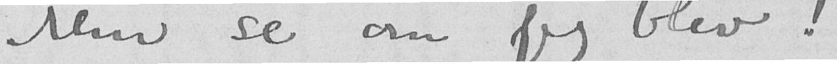Men se om jeg blev!
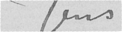Jens
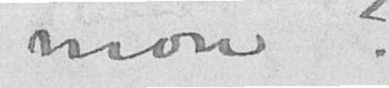mon?
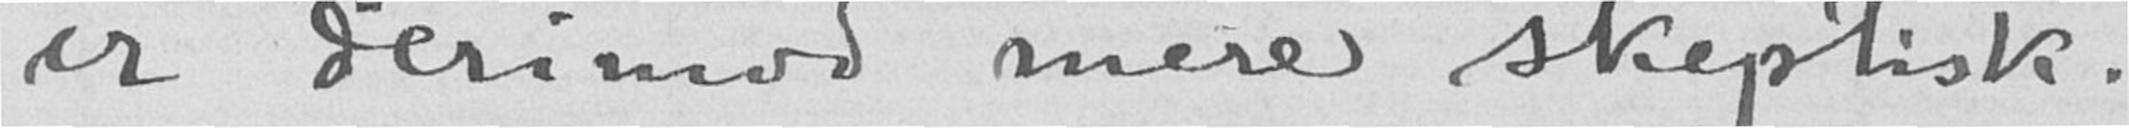er derimod mere skeptisk.
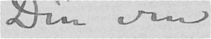Din ven
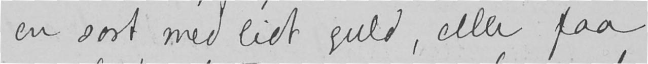en sort med lidt guld, eller faa
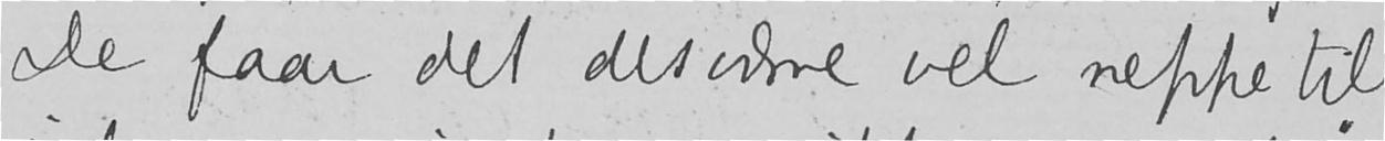De faar det desværre vel neppe til
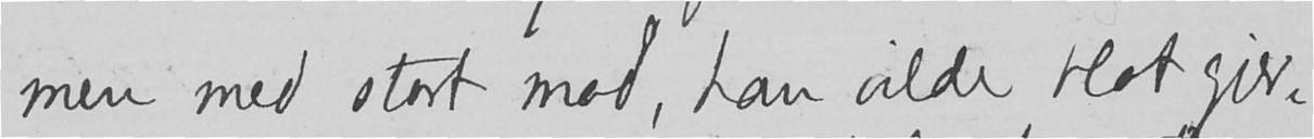men med stort mod, han vilde blot gjer-
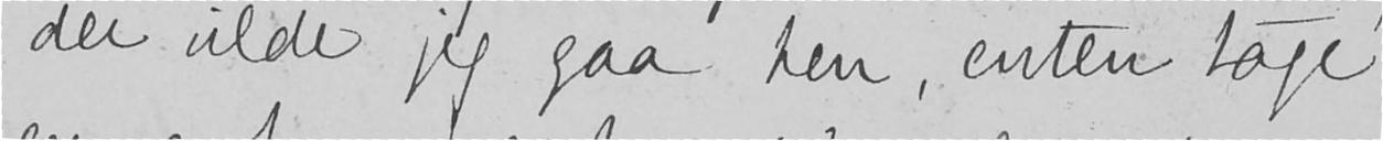der vilde jeg gaa hen, enten tage
